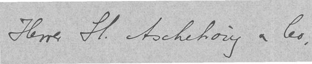Herrer H. Aschehoug & Co,
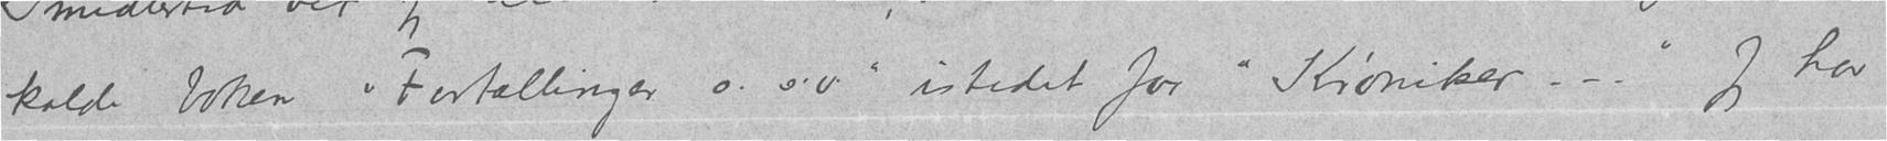kalde boken «Fortællinger o.s.v.» istedet for "Krøniker – –." Jeg har
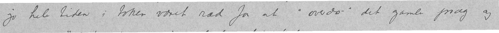jo hele tiden i boken været ræd for at «overdo» det gamle præg og
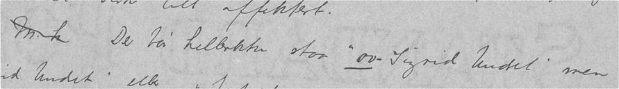M.h. Der bør hellerikke staa "av Sigrid Undset" men
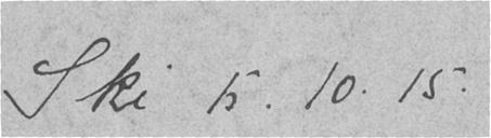Ski 1.10.15.
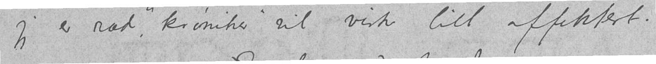jeg er ræd "krøniker" vil virke litt affektert.
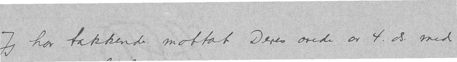Jeg har takkende mottat Deres orede av 4.ds. med
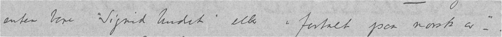enten bare "Sigrid Undset" eller "fortalt paa norsk av –".
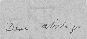Dere ærbødige
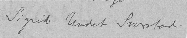Sigrid Undset Svarstad .
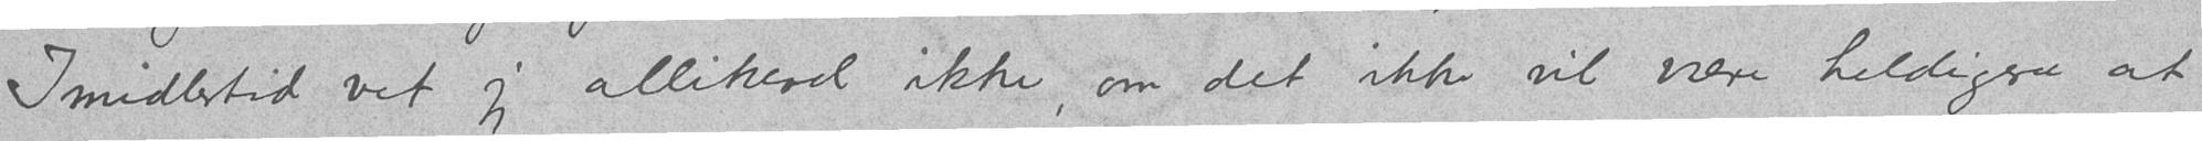Imidlertid vet jeg allikevel ikke, om det ikke vil være heldigere at
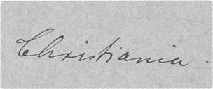Christiania.
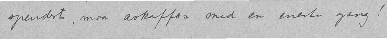spendert, maa avskaffes med en eneste gang!
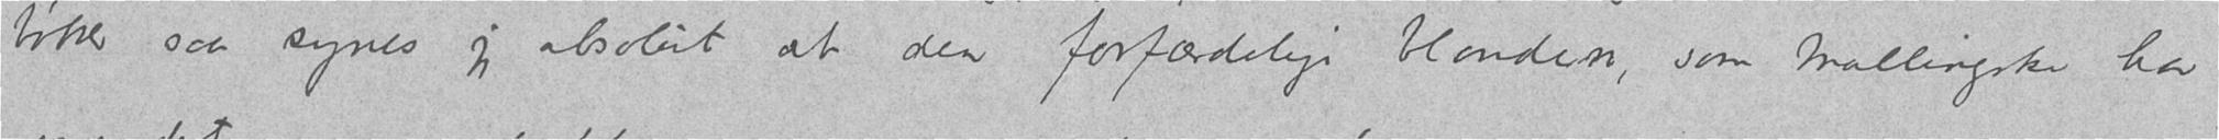bøker saa synes jeg absolut at den forfærdelige blonden, som Mallingske har
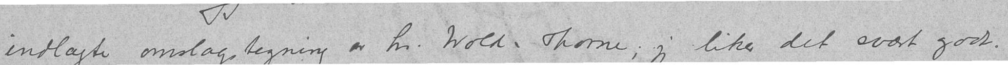indlagte omslagstegning av hr. Wold-Thorne; jeg liker det svært godt.
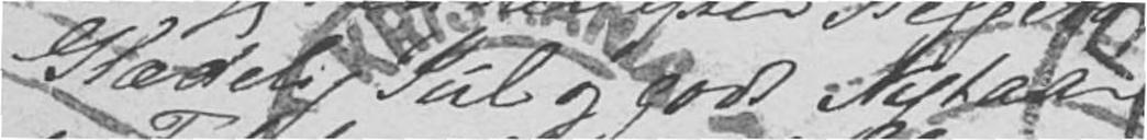Glædelig Jul og godt Nytaar
